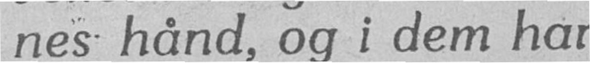nes hånd, og i dem har
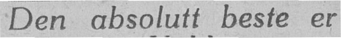Den absolutt beste er
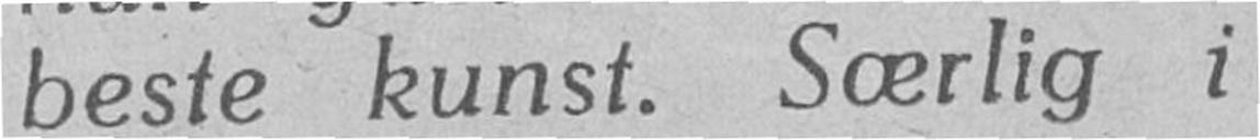beste kunst. Særlig i
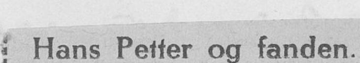Hans Petter og fanden.
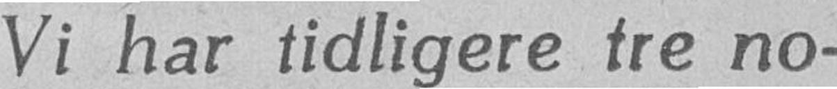Vi har tidligere tre no-
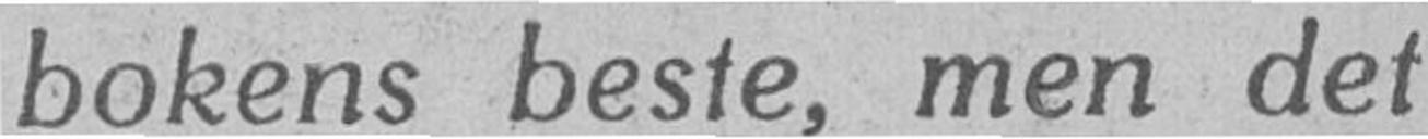bokens beste, men det
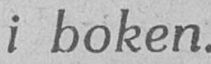i boken.
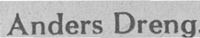Anders Dreng.
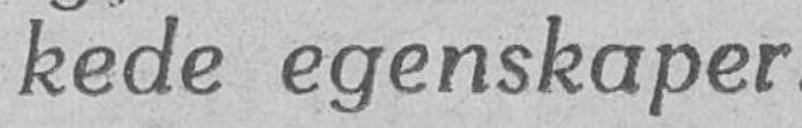kede egenskaper.
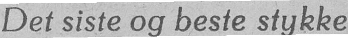Det siste og beste stykke
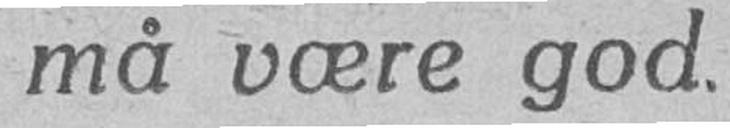må være god.
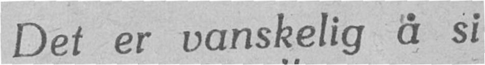Det er vanskelig å si
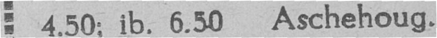4.50; ib. 6.50 Aschehoug.
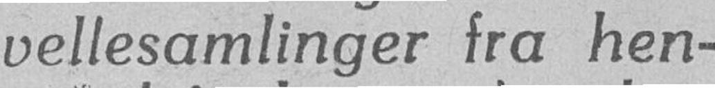vellesamlinger fra hen-
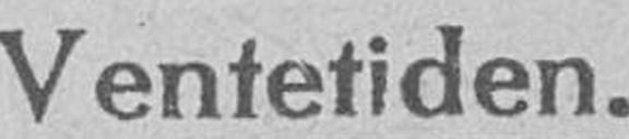Ventetiden.
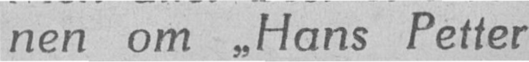nen om "Hans Petter
